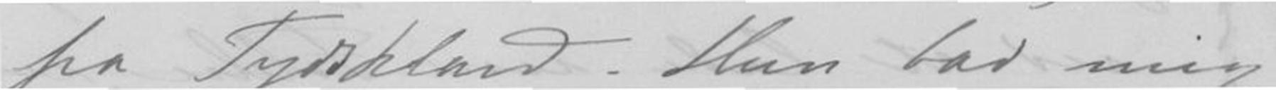fra Tyskland. Hun bad mig
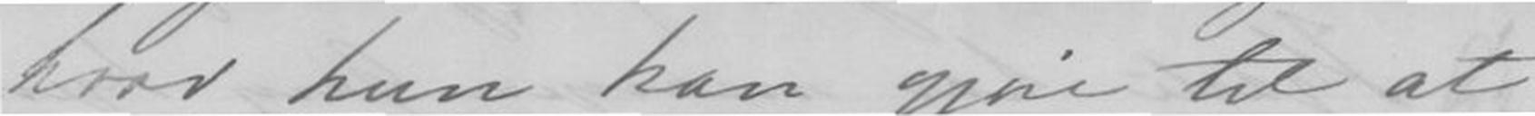tror hun kan gjøre til at
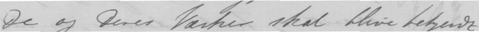De og Deres værker skal blive bekjendt
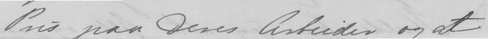Pris paa Deres Arbeider og at
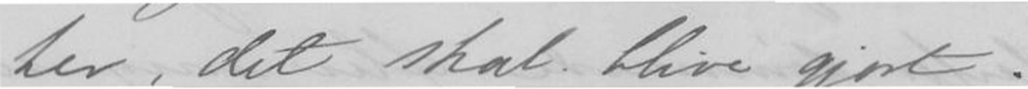der, det skal blive gjort.
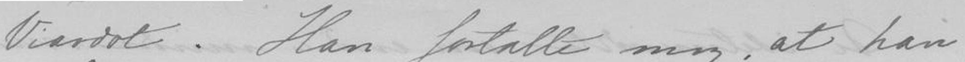Viardot. Han fortalte mig at han
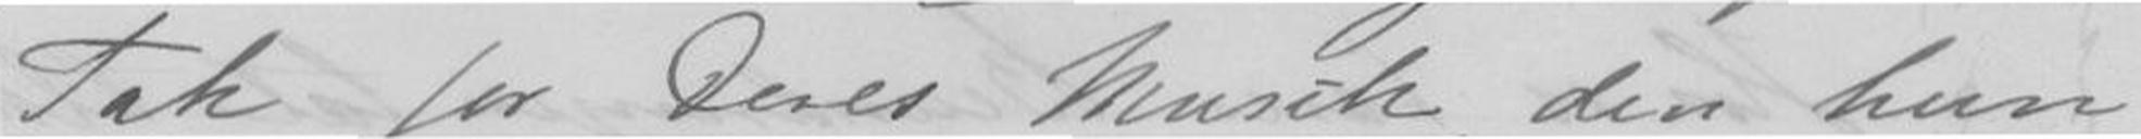Tak for Deres Musik, der hun
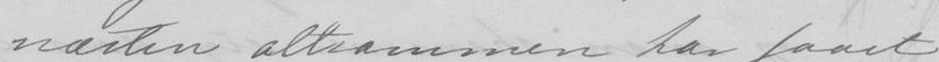næsten altsammen har faaet
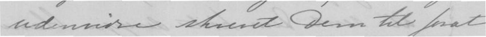udenvidere skrevet Dem til forat
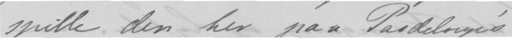spille den her paa Pasdelovge's
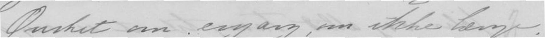Ønsket om engang, om ikke længe,
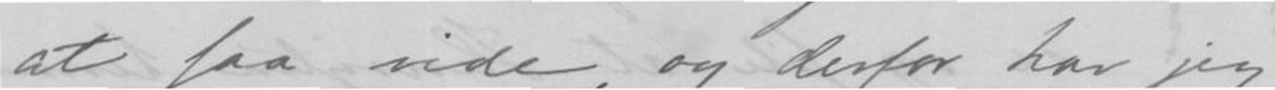at faa vide, og derfor har jeg
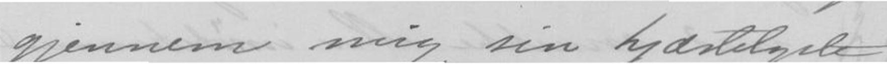gjennem mig sin hjærteligste
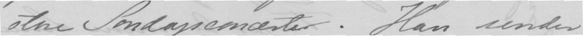Store Søndagsconcerter. Han sender
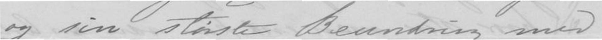og sin største Beundring med
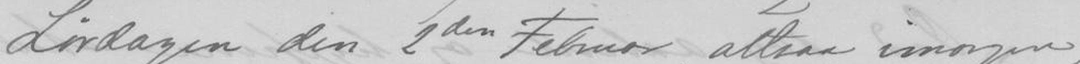Lørdagen den 2den Februar, altsaa imorgen,
